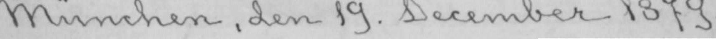München, den 19. December 1879.
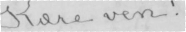Kære ven!
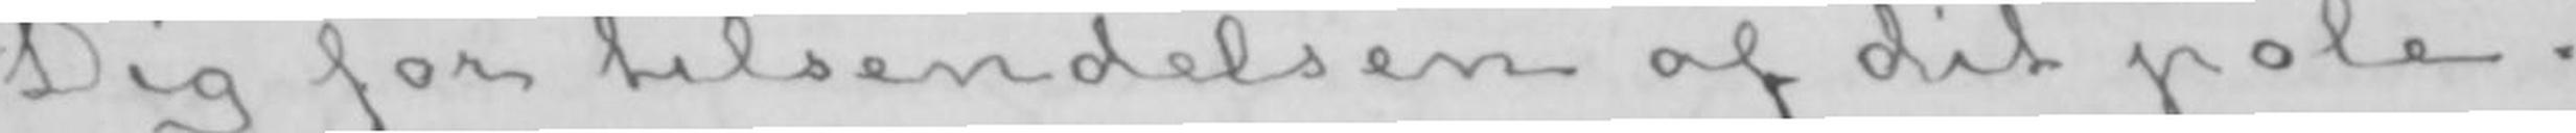Dig for tilsendelsen af dit pole-
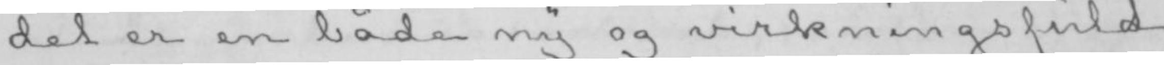det er en både ny og virkningsfuld
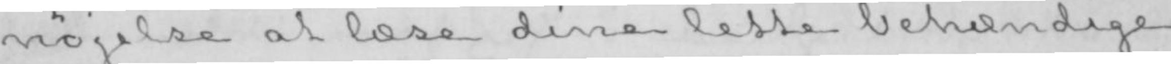nøjelse at læse dine lette behændige
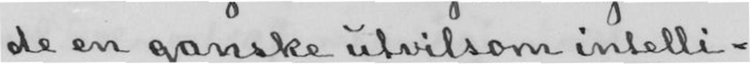de en ganske utvilsom intelli-
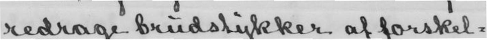redrage brudstykker af forskel-
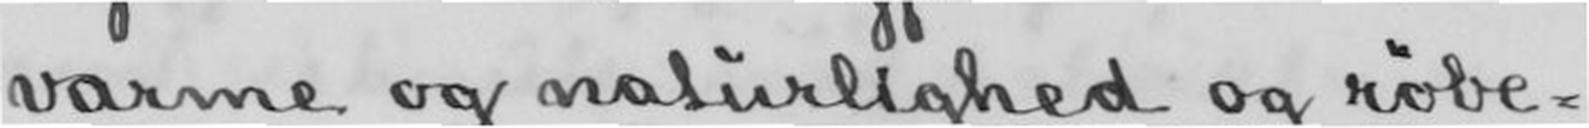varme og naturlighed og røbe-
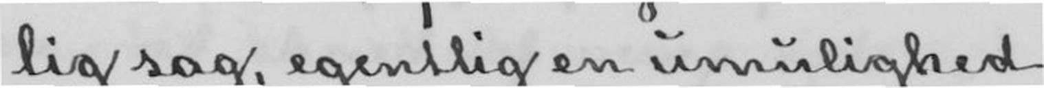lig sag, egentlig en umulighed,
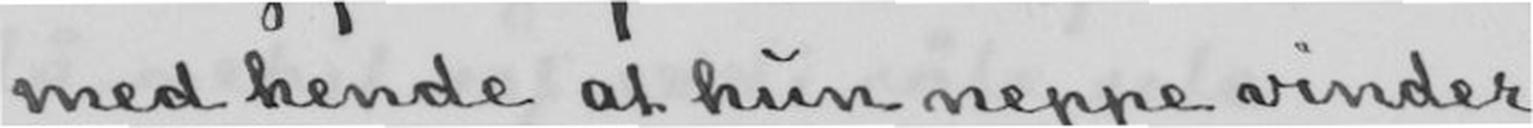med hende at hun neppe vinder
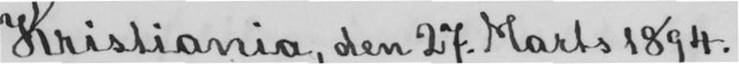Kristiania, den 27. Marts 1894.
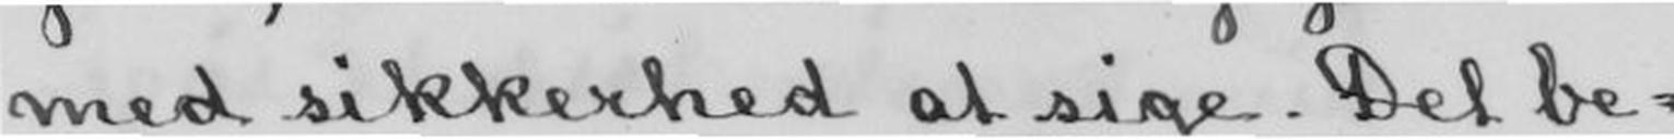med sikkerhed at sige. Det be-
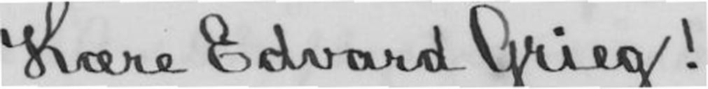Kære Edvard Grieg!
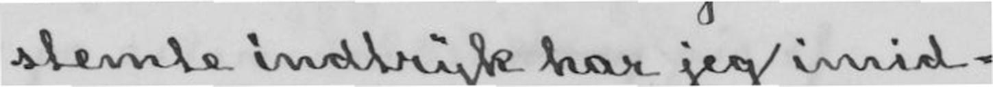stemte indtryk har jeg imid-
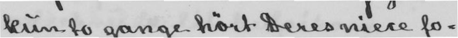kun to gange hørt Deres niece fo-
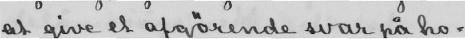at give et afgørende svar på ho-
